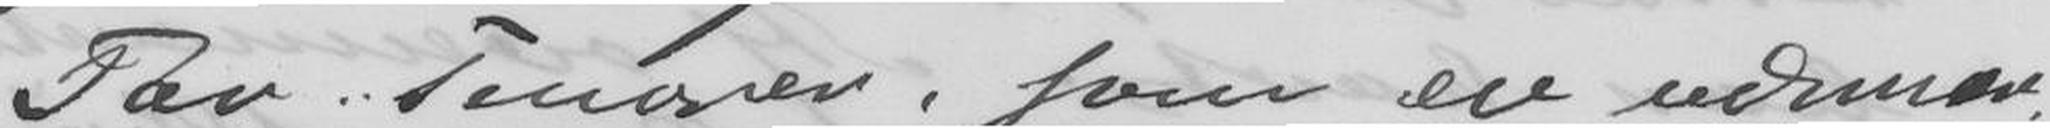Par Tenorer, som er udmær-
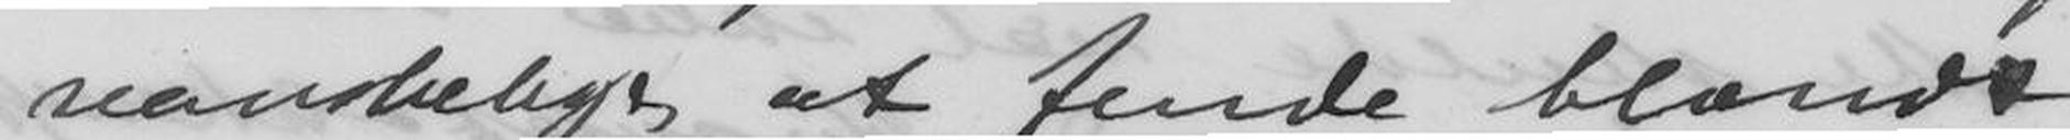vanskelig at finde blandt
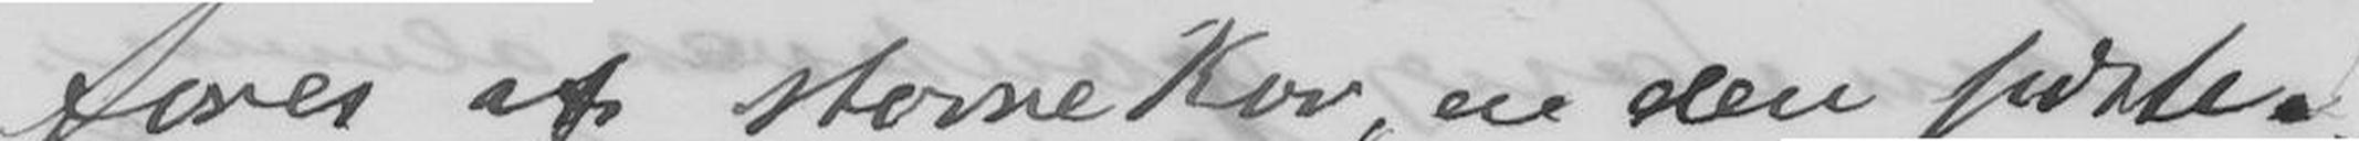føres af store Kor, er den sidste.
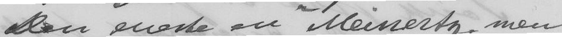Den eneste er Meinertz, men
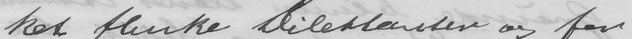ket flinke Dilettanter og for
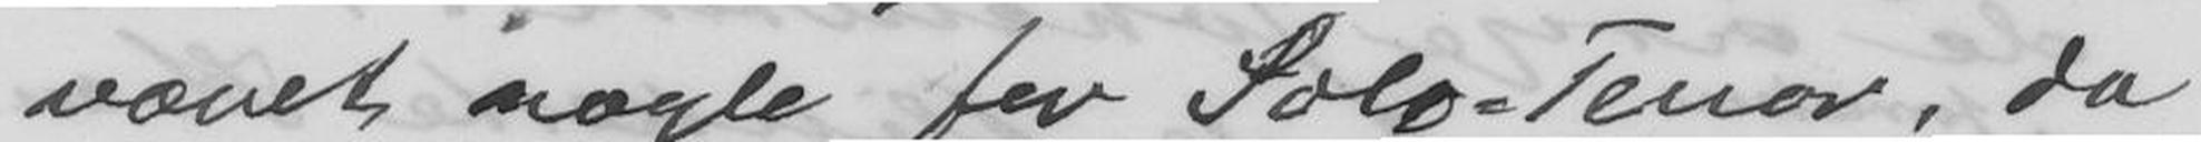været nogle for Solo-Tenor, da
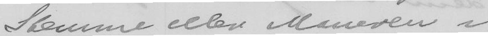Stemme eller Maneren i
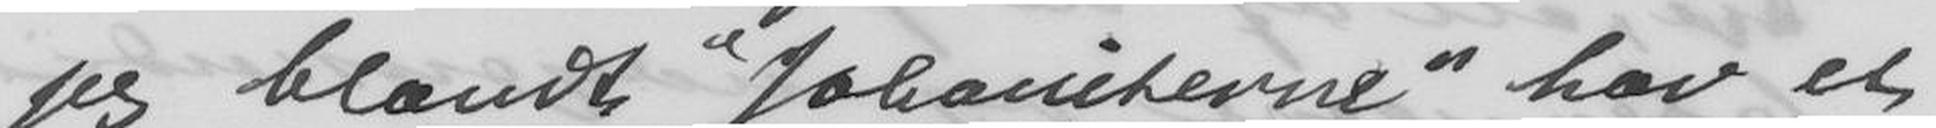jeg blandt "Johaniterne" har et
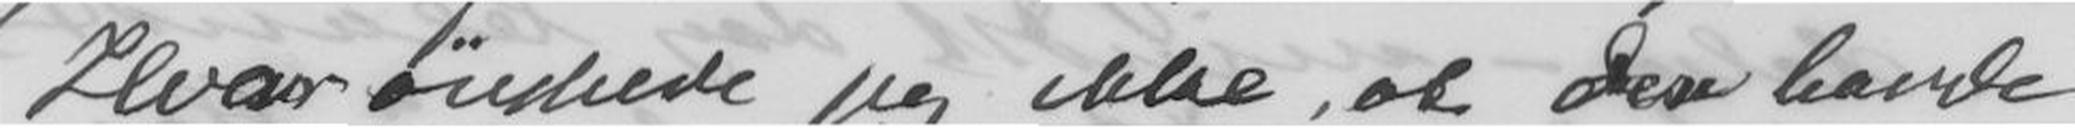Hvor ønskede jeg ikke, at der havde
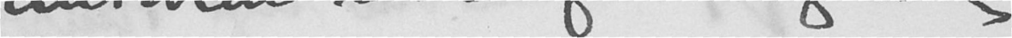antiken eller fra engelsk
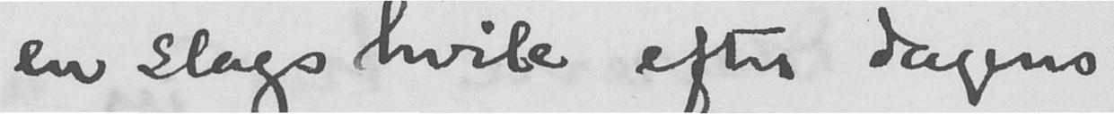en slags hvile efter dagens
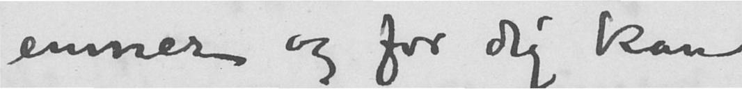emner og for dig kan
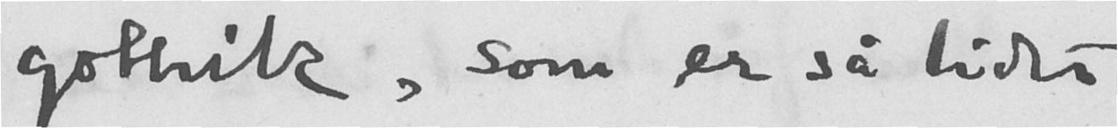gothik, som er så lidet
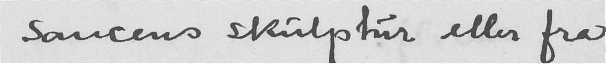sancens skulptur eller fra
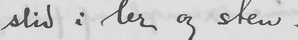slid i ler og sten.
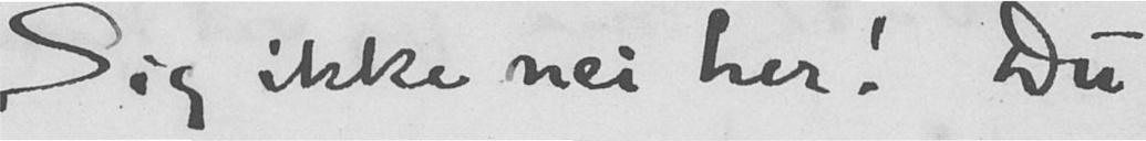Sig ikke nei her! Du
